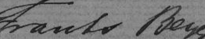Frants Beyer
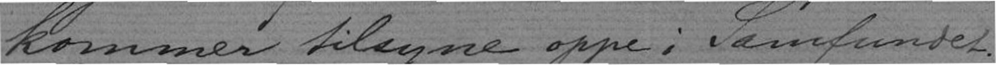kommer tilsyne oppe i Samfundet.
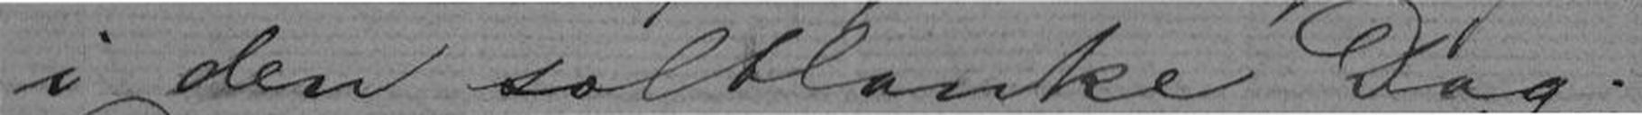i den solblanke Dag. -
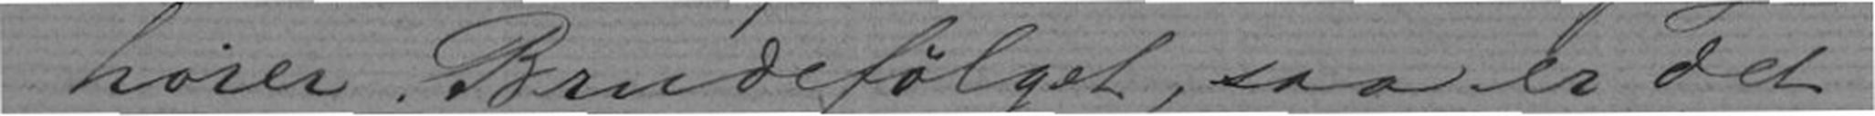hører Brudefølget, saa er det
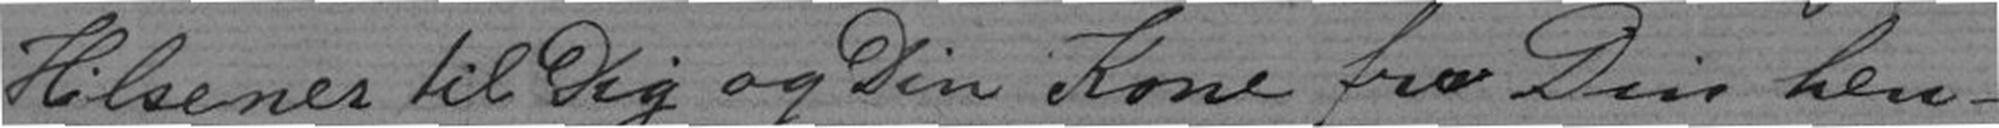Hilsener til Dig og Din Kone fra Din hen-
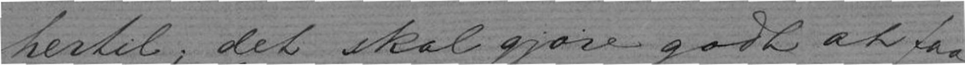hertil; det skal gjøre godt at faa
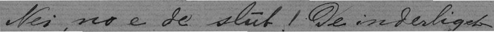Nei, no e de' slut! De inderligste
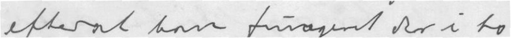efterat have fungeret der i to
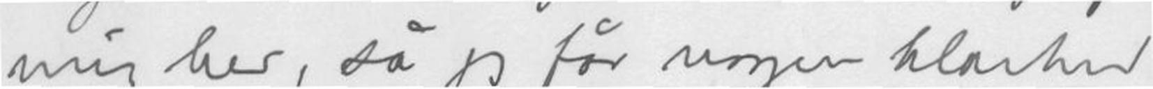mig her, så jeg får nogen klarhed
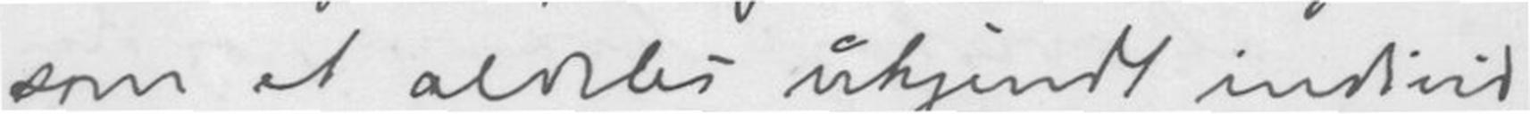som et aldeles ukjende individ
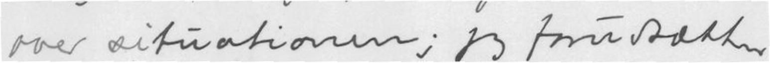over situationen; jeg forutsætter
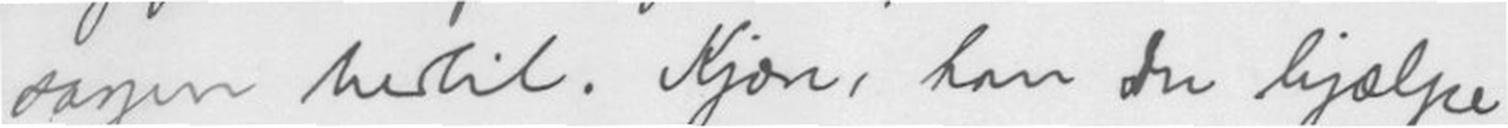sagen hertil. Kjære, kan du hjælpe
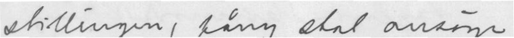stillingen, påny skal ansøge
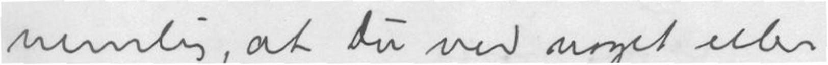nemlig, at du ved noget eller
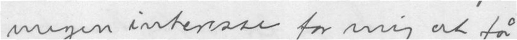megen interesse for mig at få
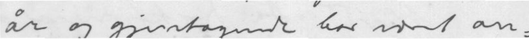år og gjentagende har været an-
